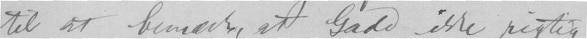til at bemærke, at Gade ikke rigtig
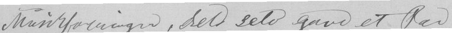Musikforeningen, dels selv gave et Par
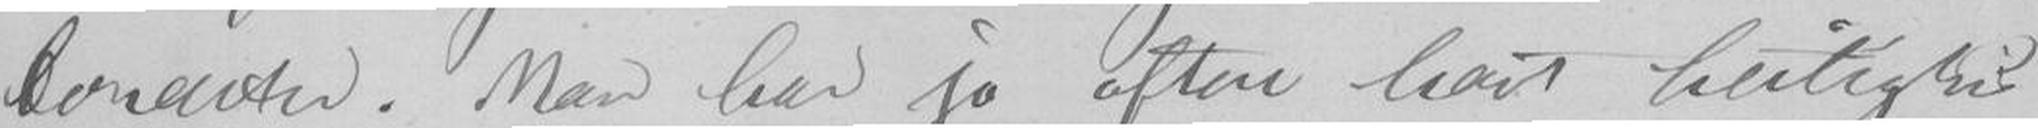Concerter. Man har jo oftere havt Leilighed
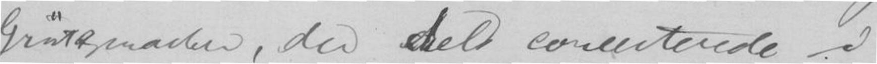Grüntzmacher, der dels concerterede i
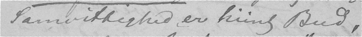Samvittighed er hiint Bud,
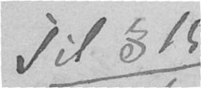Til § 15
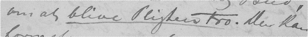om at blive Pligten tro. Den kan
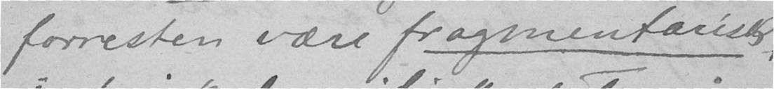forresten være fragmentarisk,
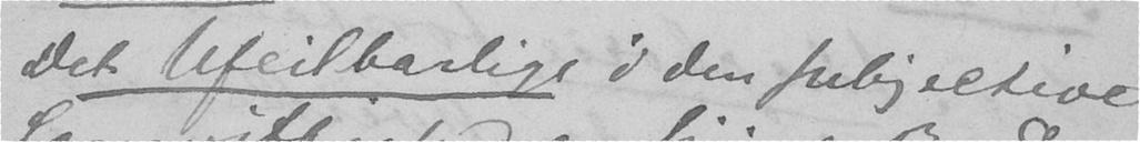Det ufeilbarlige i den subjective
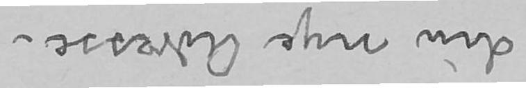din nye Adresse.
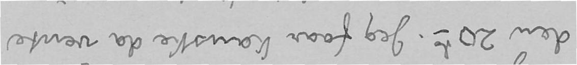den 20de. Jeg faar kanske da vente
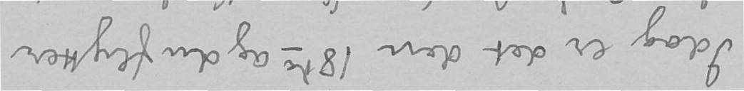Idag er det den 18de og du flytter
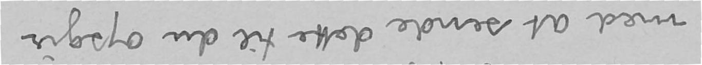med at sende dette til du opgir
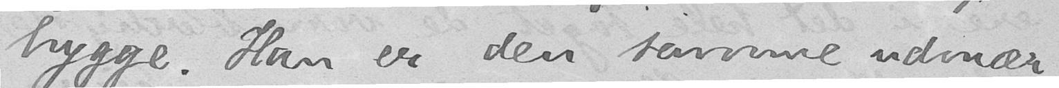hygge. Han er den samme udmær-
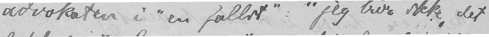advokaten i "en fallit": "jeg tror ikke, det
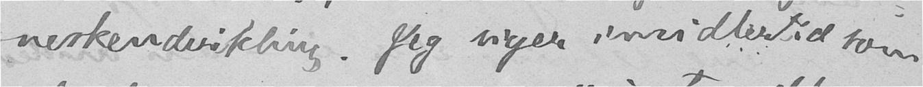neskeudvikling. Jeg siger imidlertid som
